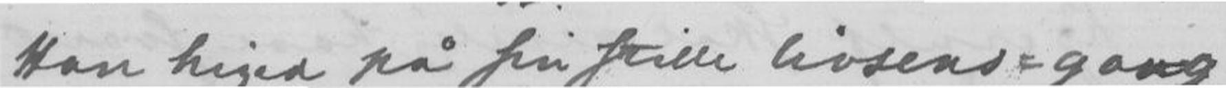Han higed på sin stille livsens=gang
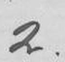2.
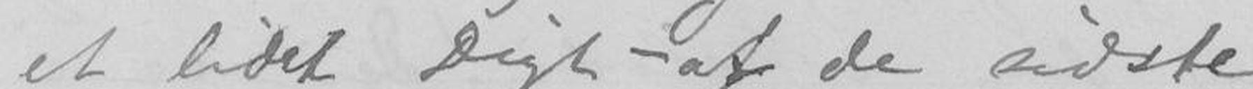et lidet Digt - af de sidste
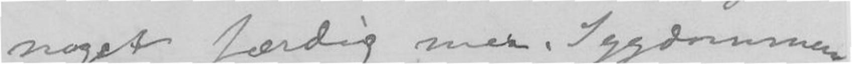noget færdig mer. Sygdommen
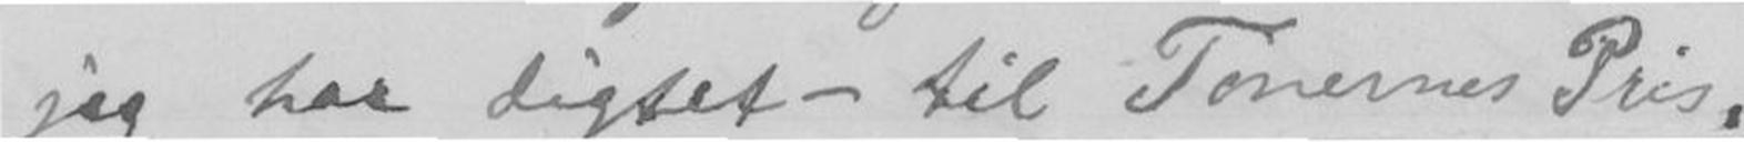jeg har digtet- til Tonernes Pris.
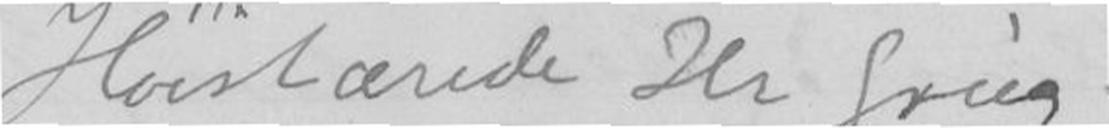Høistærede Hr Grieg.
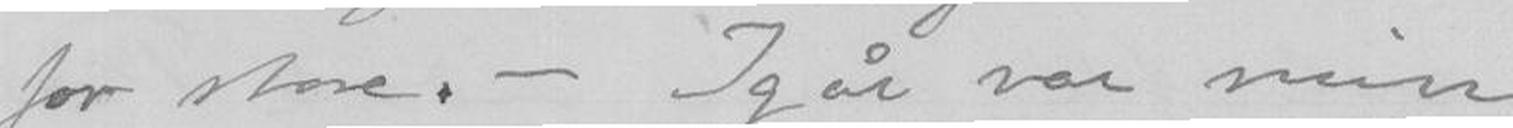for store. - Igår var min
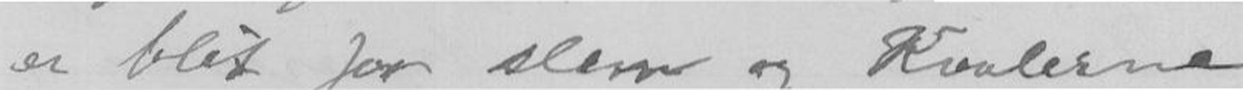er blit for slem og Kvalerne
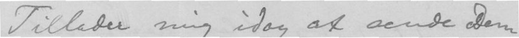Tillader mig idag at sende Dem
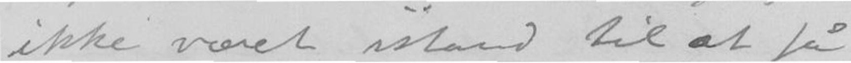ikke været istand til at få
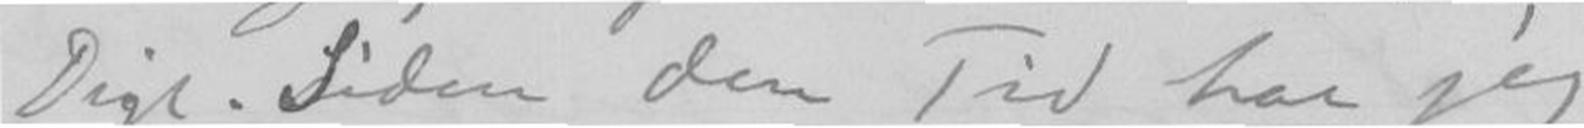Digt. Siden den Tid har jeg
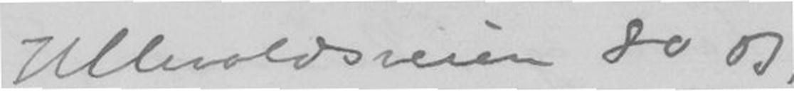Ullevoldsveien 80 B
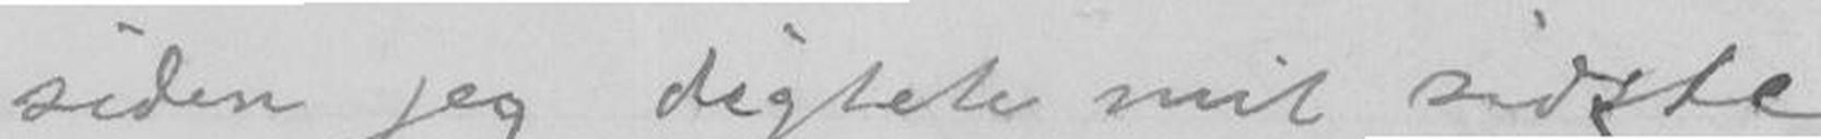siden jeg digtede mit sidste
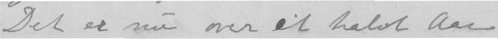Det er nu over et halvt Aar
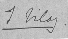1 bilag.
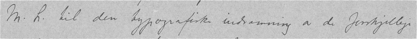M.h. til den typografiske indramning av de forskjellige
